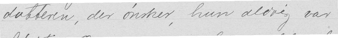datteren, der ønsker, hun aldrig var
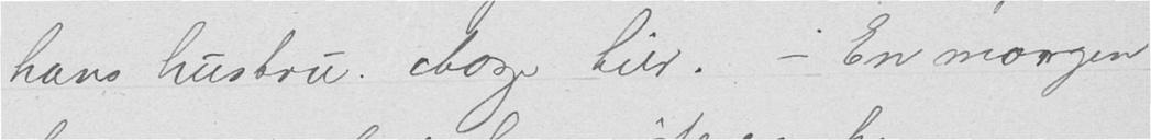hans hustru. Aage tier. - En morgen
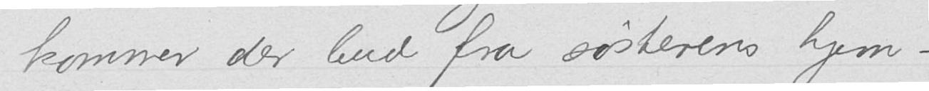kommer der bud fra søsterens hjem -
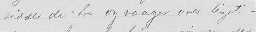sidder de tre og vaager over liget –
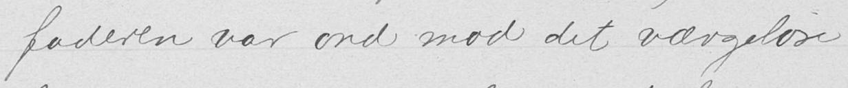faderen var ond mod det værgeløse
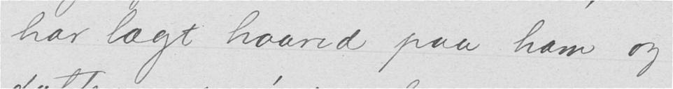har lagt haand paa ham og
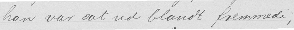han var sat ud blandt fremmede,
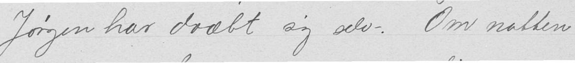Jørgen har dræbt sig selv -. Om natten
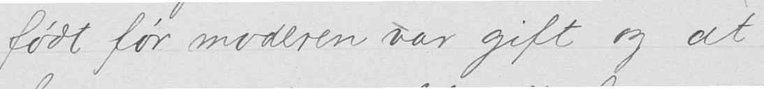født før moderen var gift og at
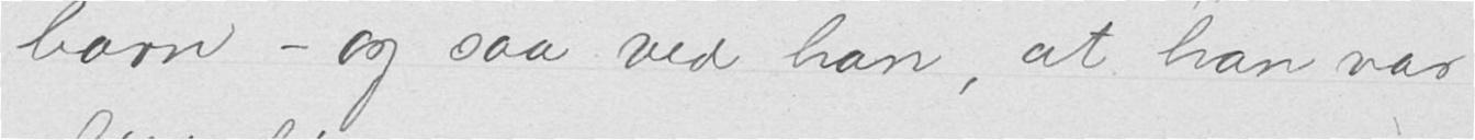barn - og saa ved han, at han var
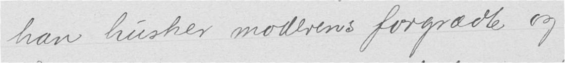han husker moderens forgrædte og
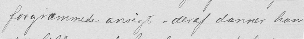forgræmmede ansigt - deraf danner han
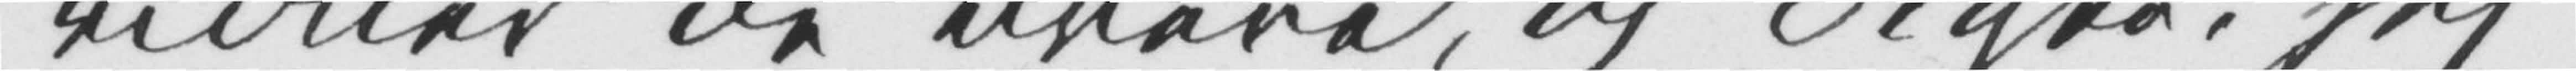vidner de Breve og Digte, jeg
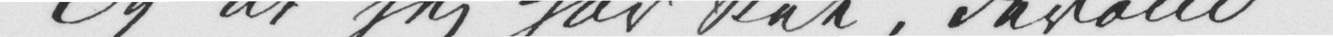Og at jeg har Ret, derom
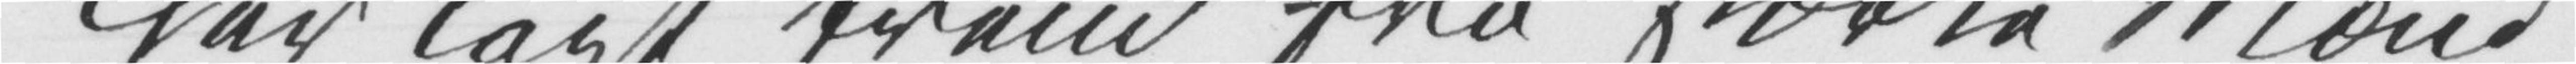har lagt frem fra «stærke Mænd
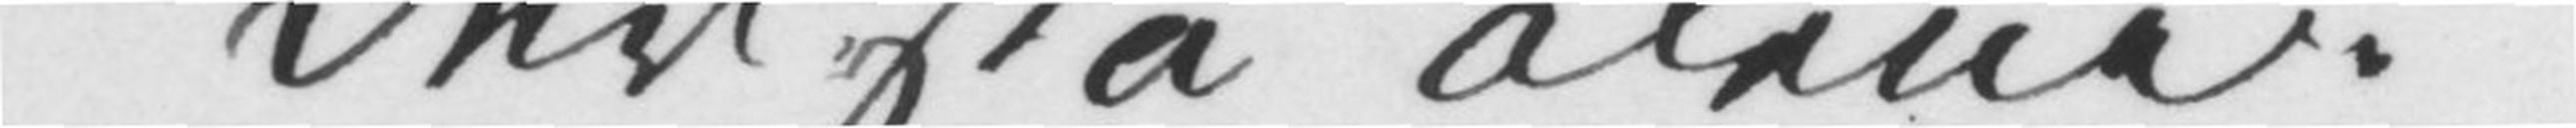der stå alene».
